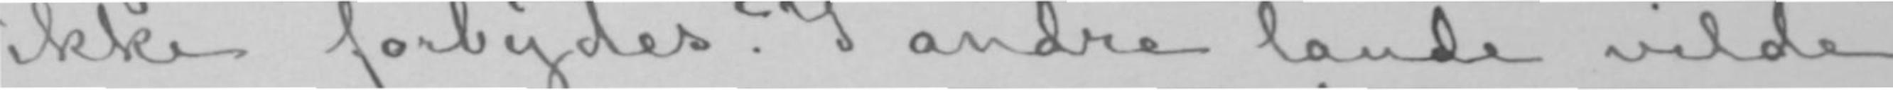ikke forbydes? I andre lande vilde
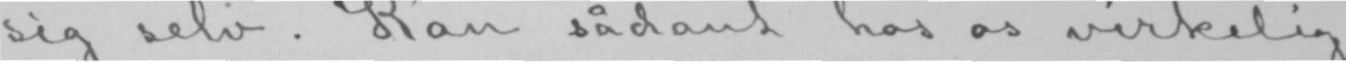sig selv. Kan sådant hos os virkelig
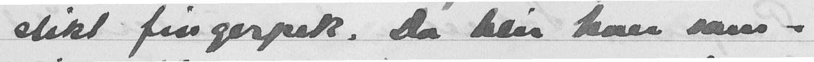slikt fingerpek. Da blir han sam-
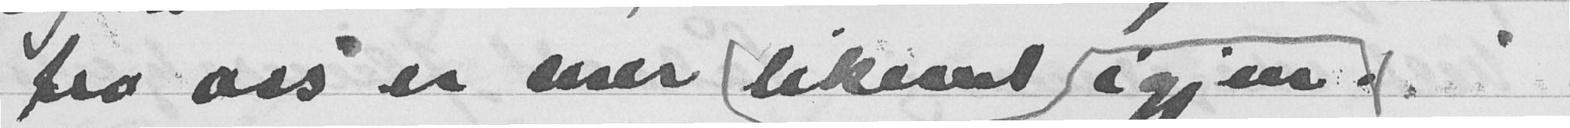fra oss er mer likevel igjen.
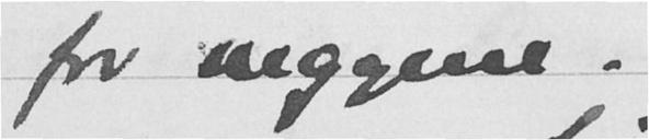for veggene.
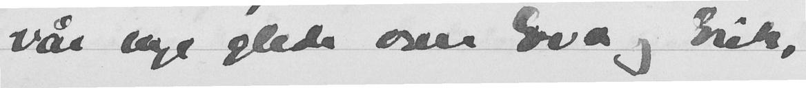vår nye glede over Eva og Erik,
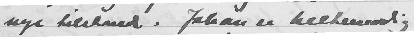nye tilsland. Johan er heltemodig
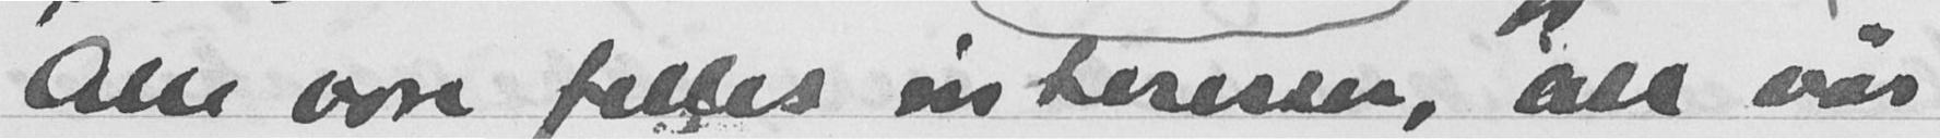Alle vore felles interesser, all vår
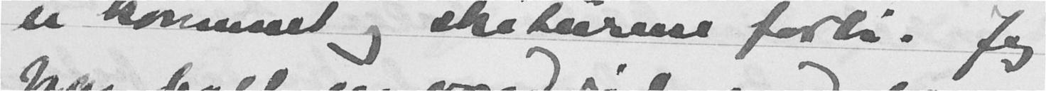er kommet og skiturene forbi. Jeg
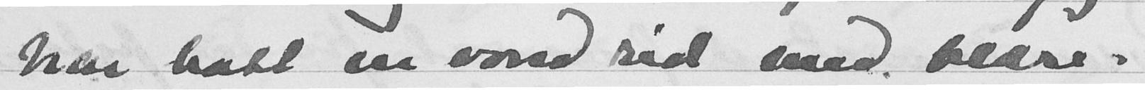her hatt en vond tid med blære-
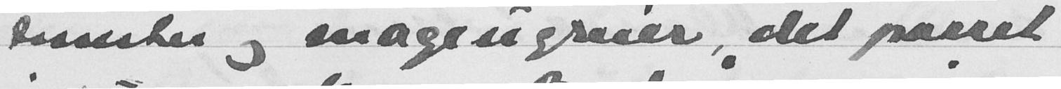smerter og mageugruier, det passet
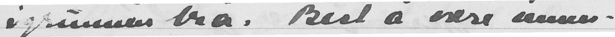igrunnenen bra. Best å være innen-
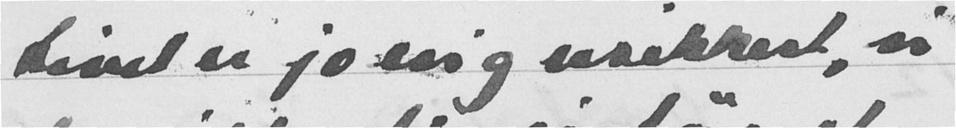Livet er jo evig usikkert, vi
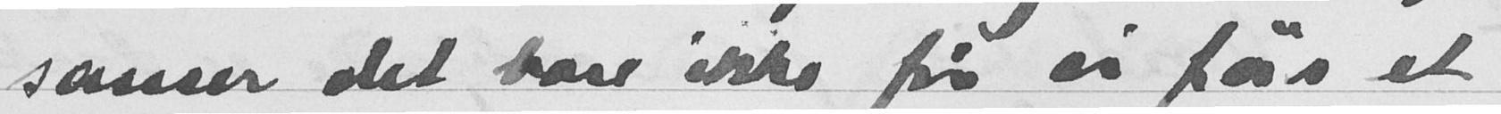sanser det bare ikke før vi får et
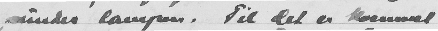under lampen. Til det er kommet
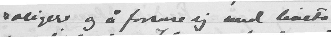roligere og å forsone sig med livets
